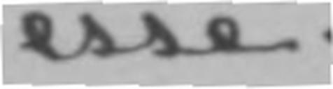esse.
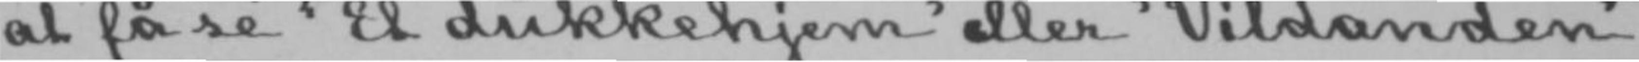at få sé «Et dukkehjem» eller «Vildanden»
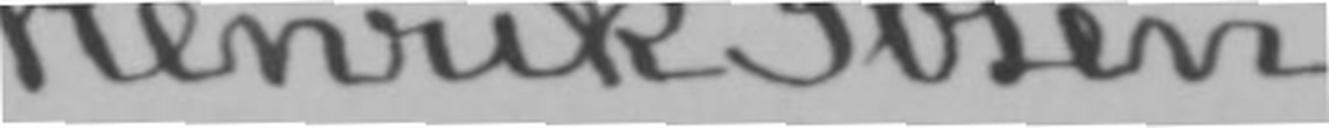Henrik Ibsen.
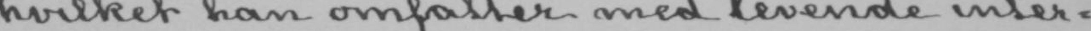hvilket han omfatter med levende inter-
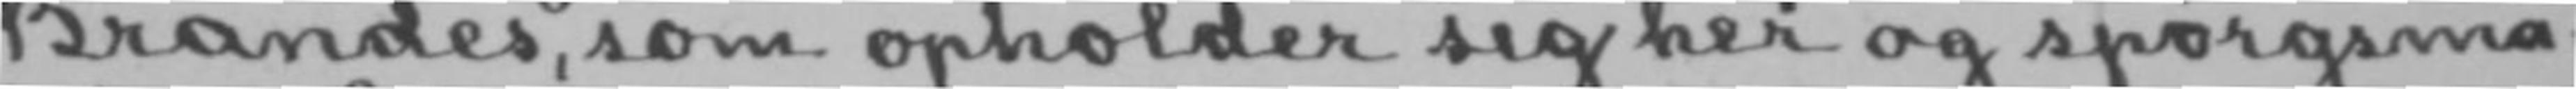Brandes, som opholder sig her og spørgsmå-
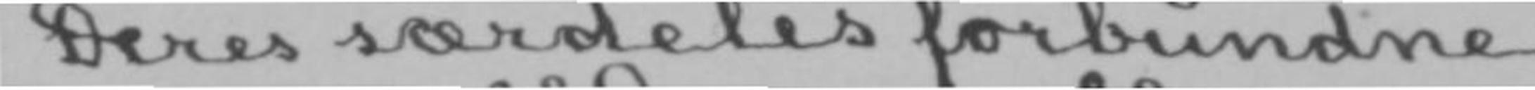Deres særdeles forbundne
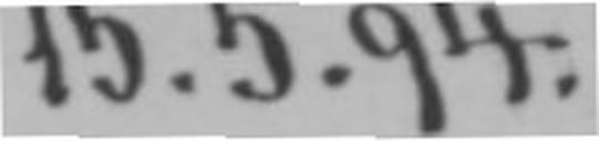15.5.94.
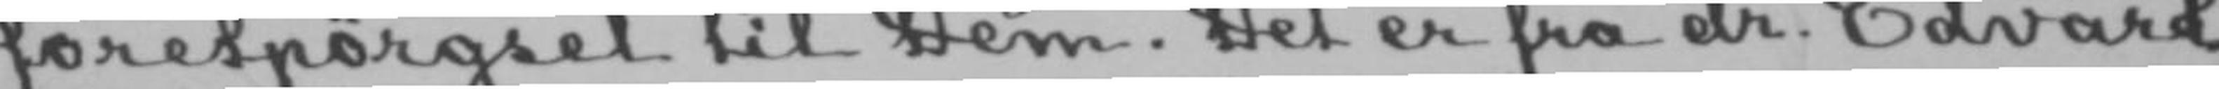forespørgsel til Dem. Det er fra dr. Edvard
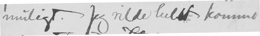muligt. Jeg vilde helst komme
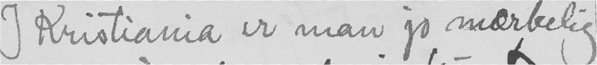I Kristiania er man jo mærkelig
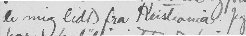le mig lidt fra Kristiania. Jeg
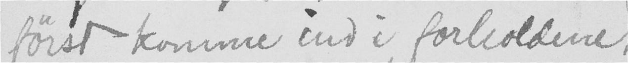først komme ind i forholdene,
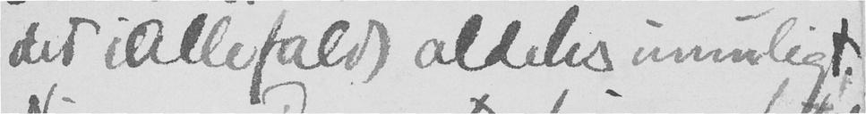det iallefald aldeles umuligt.
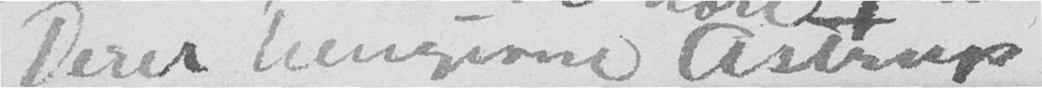Deres hengivne Astrup
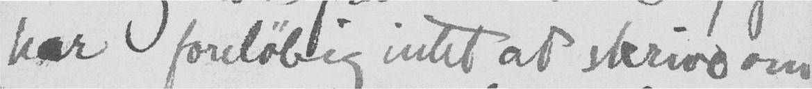har foreløbig intet at skrive om
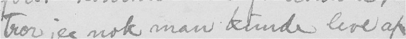tror og nok man kunde leve af
